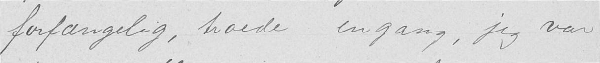forfengelig, troede engang, jeg var
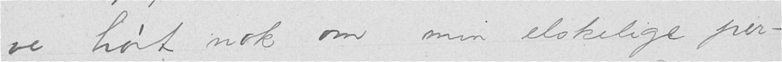ve hørt nok om min elskelige per-
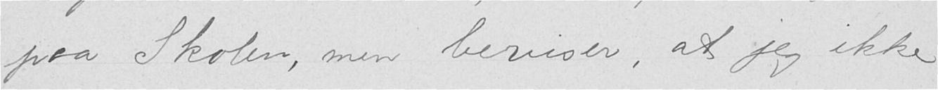paa Skolen, men beviser, at jeg ikke
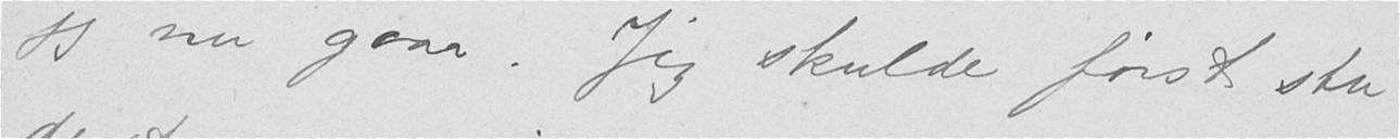jeg nu gaar. Jeg skulde først stu-
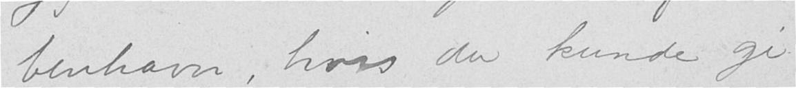benhavn, hvis du kunde gi
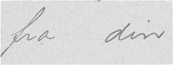fra din
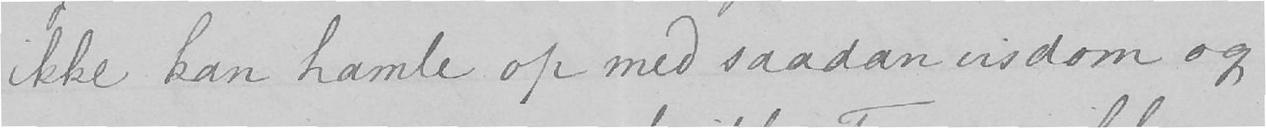ikke kan hamle op med saadan visdom og
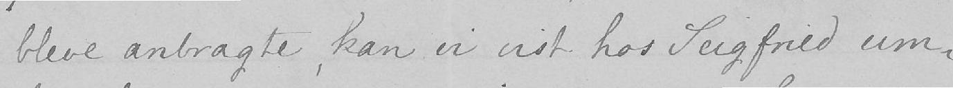bleve anbragte, kan vi vist hos Seigfried um-
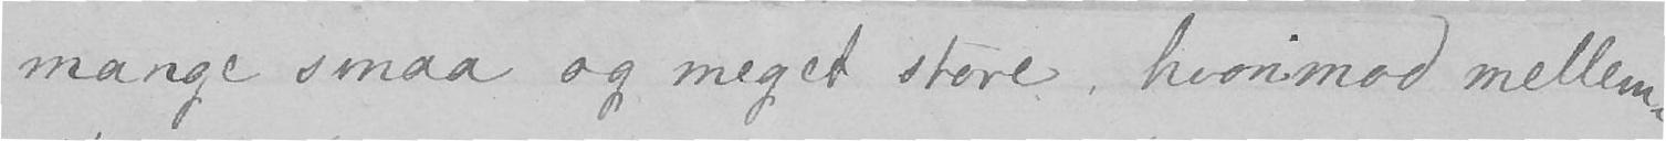mange smaa og meget store, hvorimod mellem
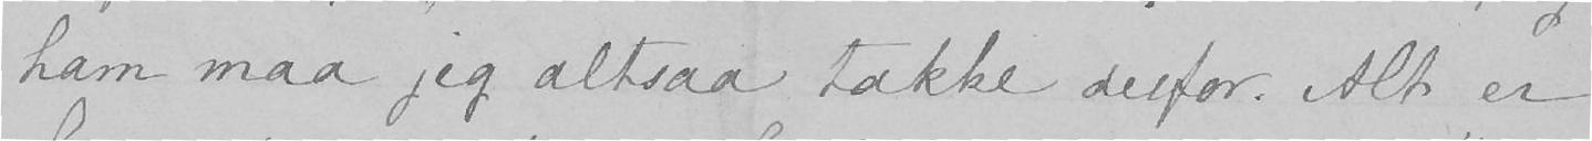ham maa jeg altsaa takke derfor. Alt er
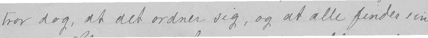tror dog, at det ordner sig, og at alle finder sin
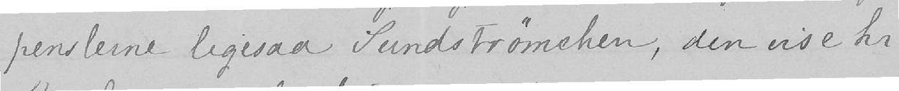penslerne ligesaa Sundstrømchen, den vise hr.
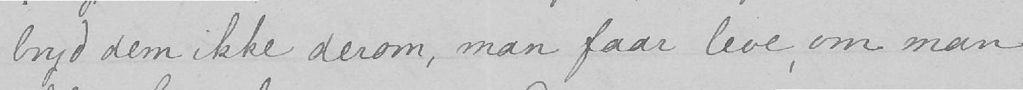bryd dem ikke derom, man faar leve, om man
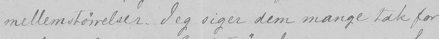mellemstørrelser. Jeg siger dem mange tak for
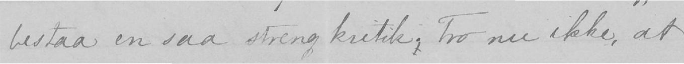bestaa en saa streng kritik. Tro nu ikke, at
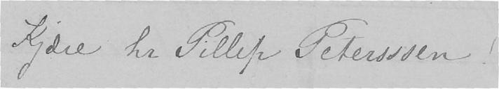Kjære hr Pillip Petersssen!
